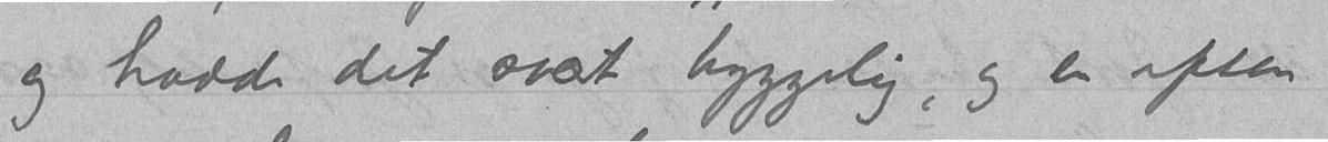og hadde det svært hyggelig, og en aften
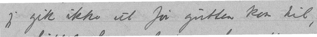jeg gik ikke ut før gutten kom til,
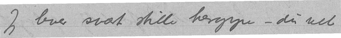Jeg lever svært stille heroppe - du vet
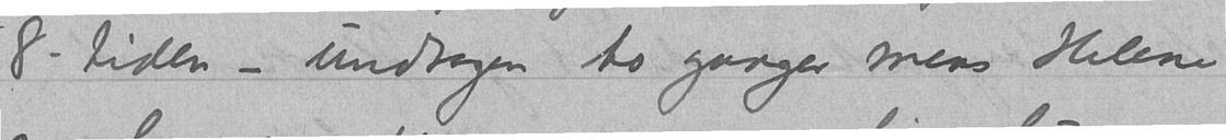8-tiden – undtagen to ganger mens Helene
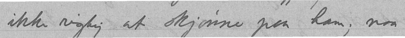ikke rigtig at skjønne paa ham; naa
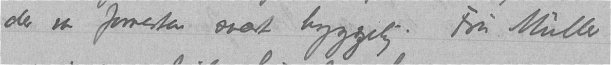der var forresten svært hyggelig. Fru Muller
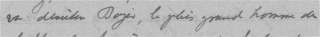var desuten Bojer, le plus grand homme des
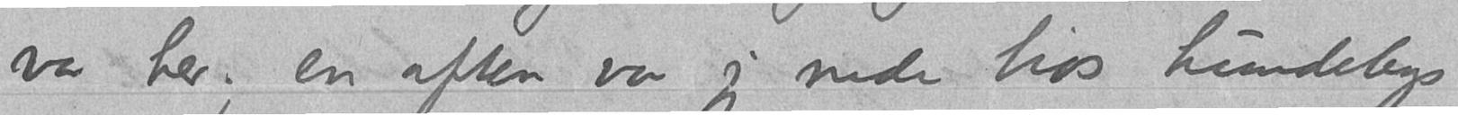var her; en aften var jeg nede hos Lundebys
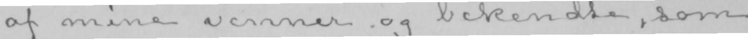af mine venner og bekendte, som
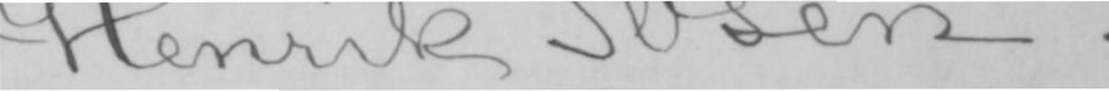Henrik Ibsen.
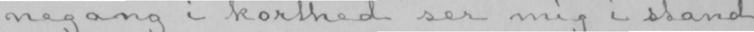negang i korthed ser mig i stand
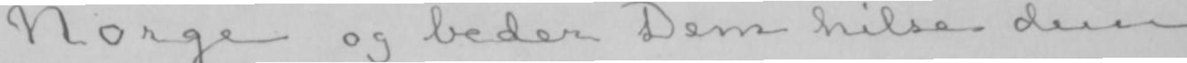Norge og beder Dem hilse dem
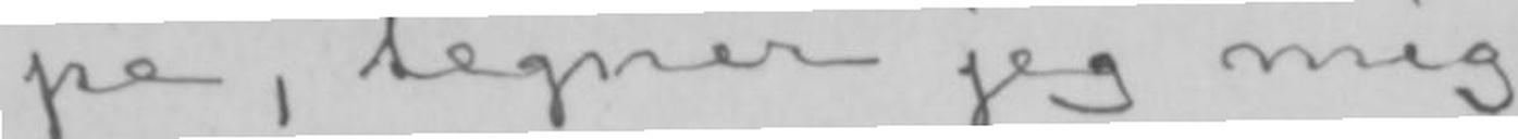pe, tegner jeg mig
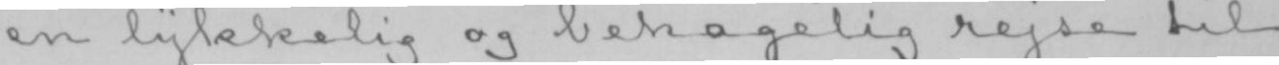en lykkelig og behagelig rejse til
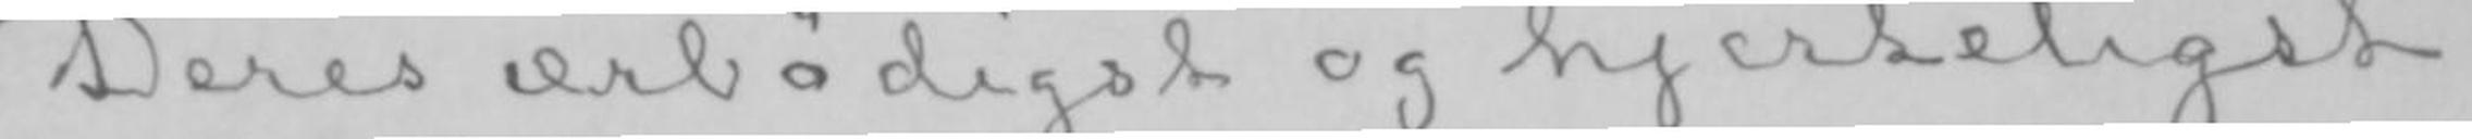Deres ærbødigst og hjerteligst
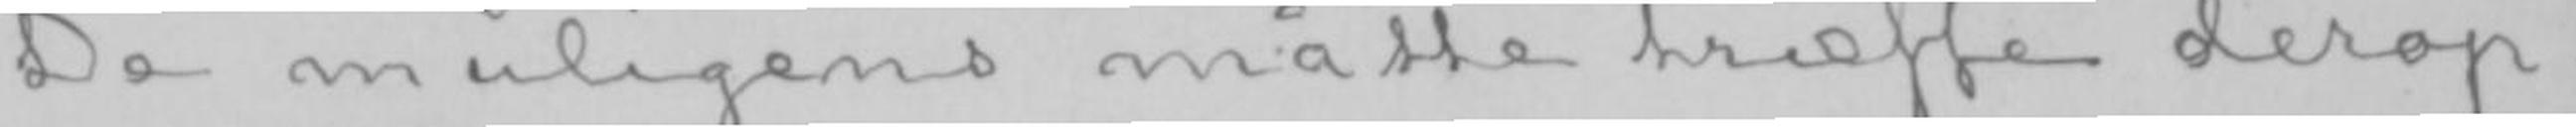De muligens måtte træffe derop-
